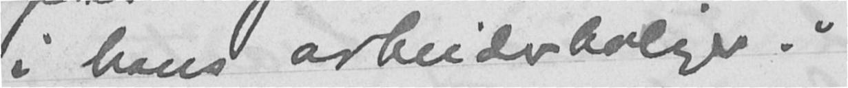i hans arbeiderboliger."
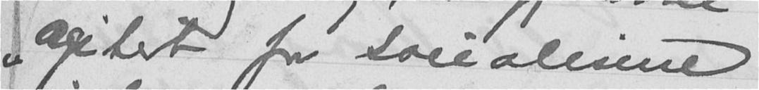"agitert for socialisme
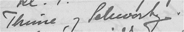Thrine og Schwartz.
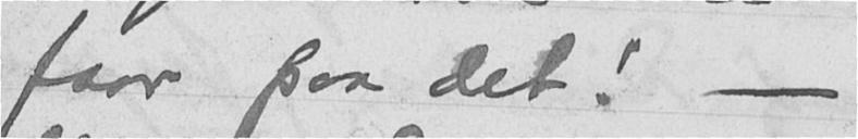faar paa det! -
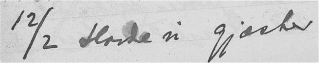12/2 Havde vi gjæster
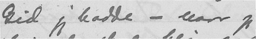Gid jeg hadde - naar jeg
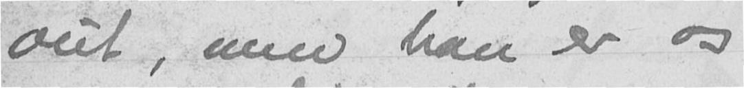out, men han er os
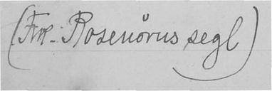(Frk. Rosenørus segl)
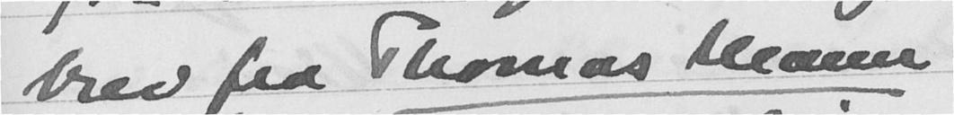brev fra Thomas Mann
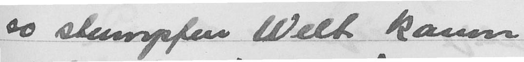so sturipfii Welt kanon
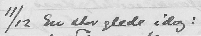11/12 En stor glede i dag:
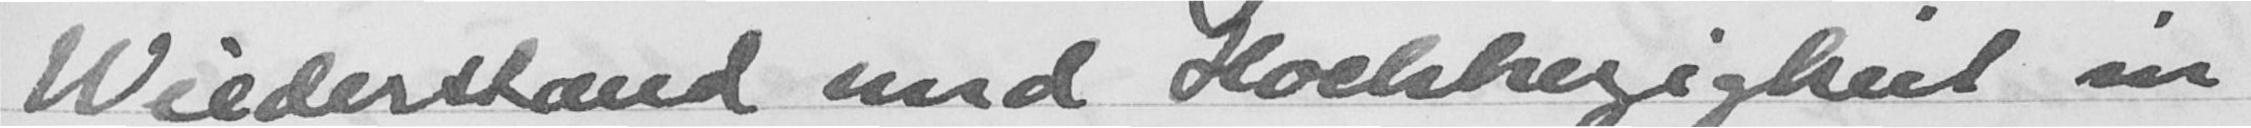Wiederstand und Hoehkergigkunt sai
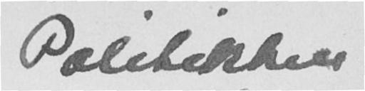Politikken
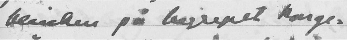blinken på begrepet konge-
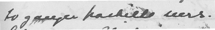to ganger fraskilt vers.
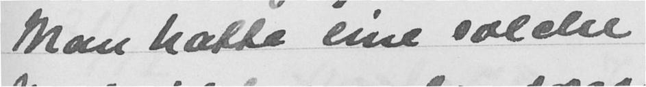Man hatte eine solche
